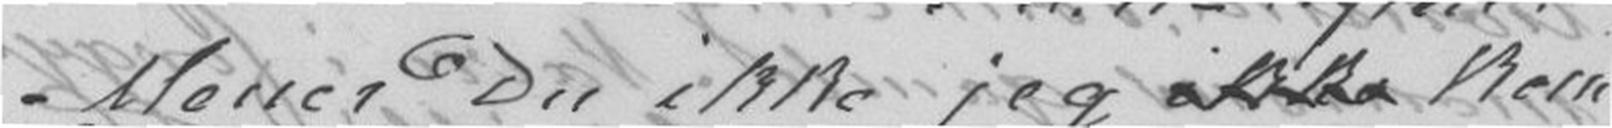Mener Du ikke jeg kom
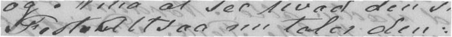Fest. Altsaa nu taler den:
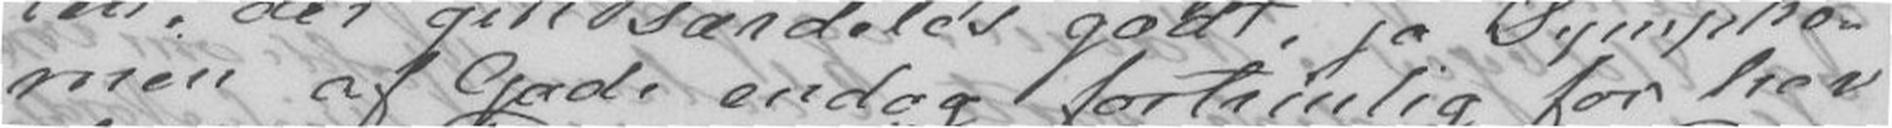nien af Gade endog fortrinlig for her
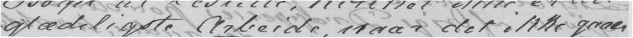glædeligste Arbeide, naar det ikke gaaer
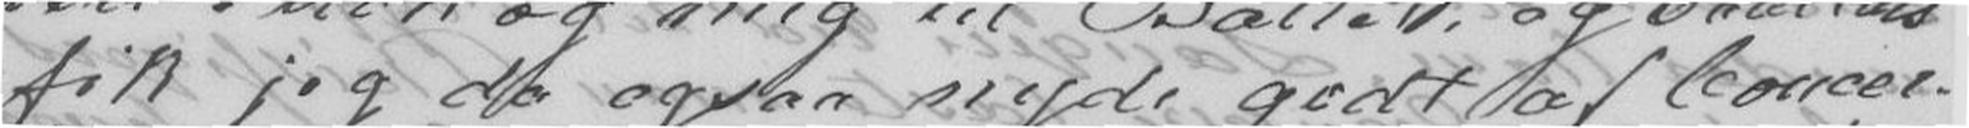fik jeg da ogsaa nyde godt af Concer-
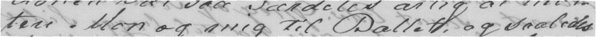tere Mor og mig til Ballet, og saaledes
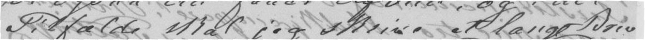Tilfælde skal jeg skrive et langt Brev
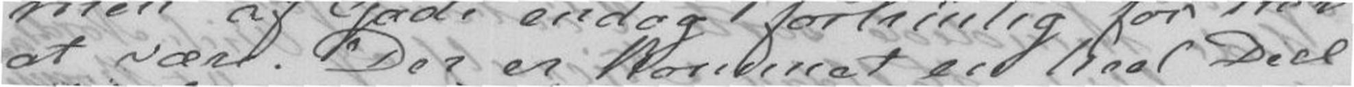at være. Der er kommet en heel Deel
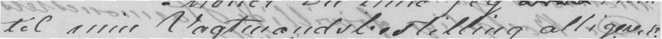til min Vagtmandsbestilling alligevel?
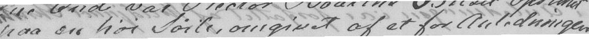paa en høi Søile, omgivet af et for Anledningen
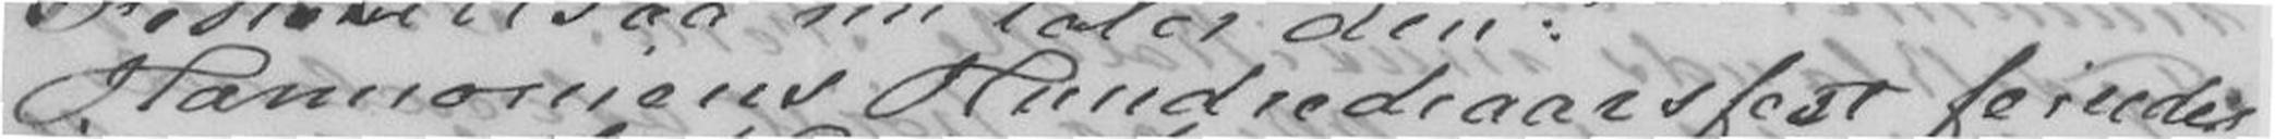Harmoniens Hundredeaarsfest feiredes
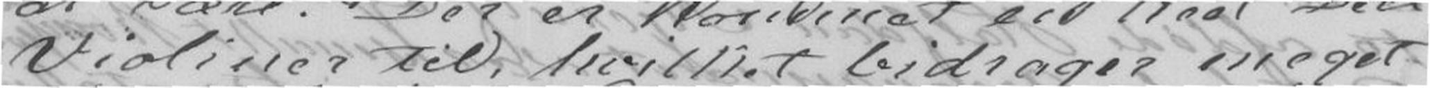Violiner til. hvilket bidrager meget
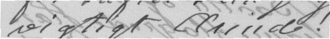vigtigt Ærinde!
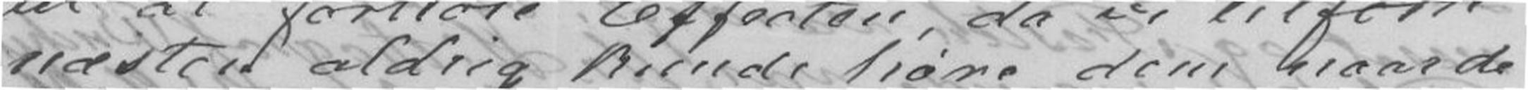næsten aldrig kunde høre dem naar de
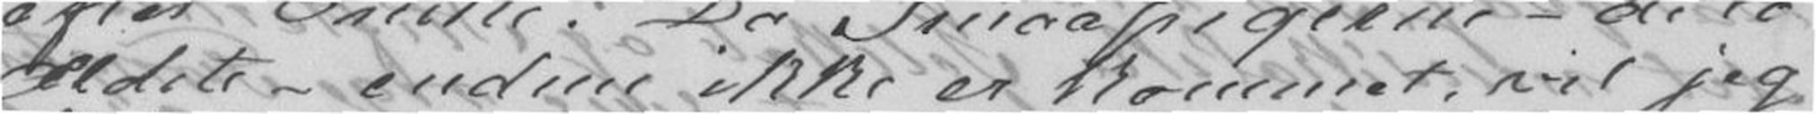Ældste - endnu ikke er kommet, vil jeg
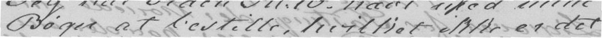Bøger at bestille, hvilket ikke er det
